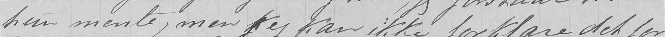hun mente, men jeg kan ikke forklare det for
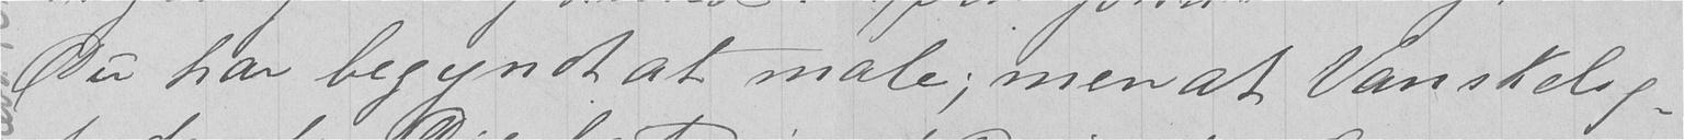Du har begyndt at male; men at Vanskelig-
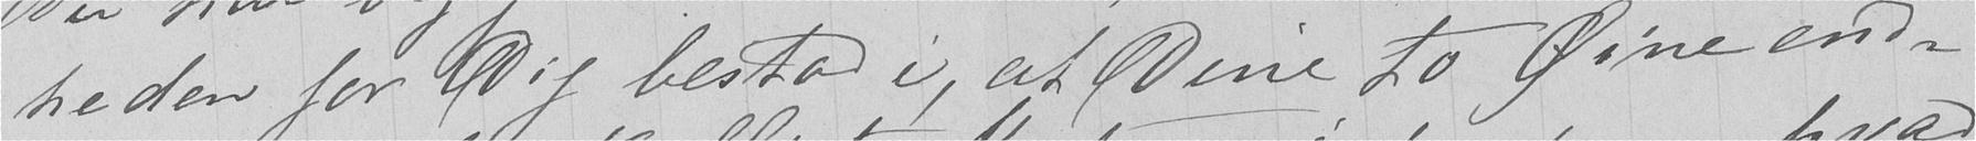heden for Dig bestod i, at Dine to Øine end-
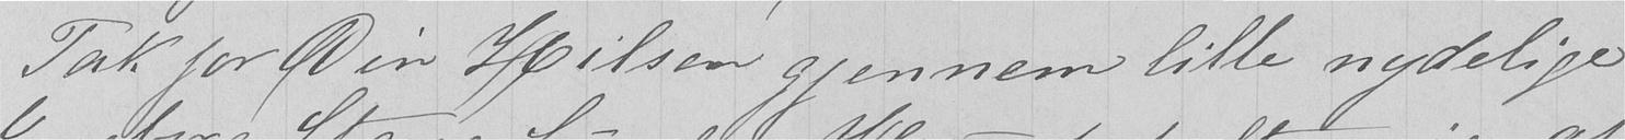Tak for Din Hilsen gjennem lille nydelige
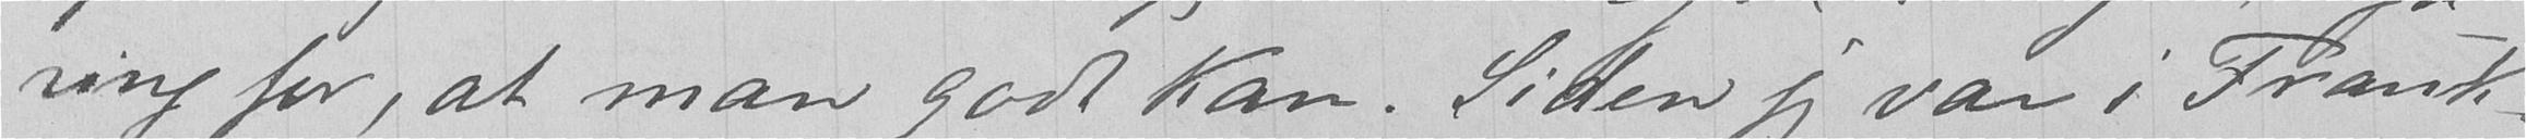ring for, at man godt kan. Siden jeg var i Frank-
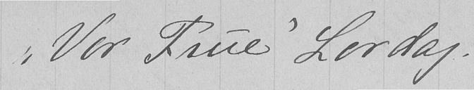"Vor Frue" Lørdag.
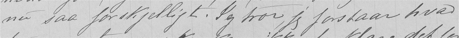nu saa forskjelligt. Jeg tror, jeg forstaar hvad
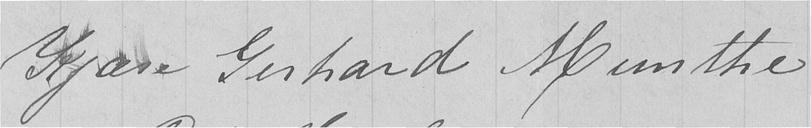Kjære Gerhard Munthe
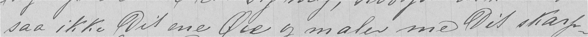saa ikke Dit ene Øie og maler med Dit skarp-
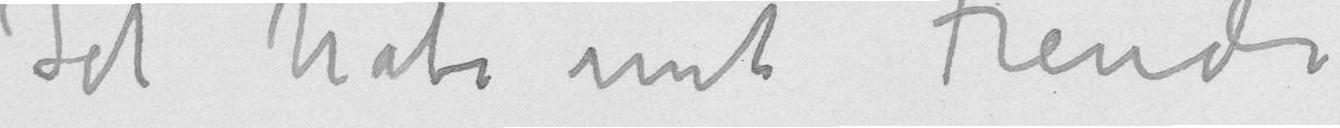Ich habe mit Freude
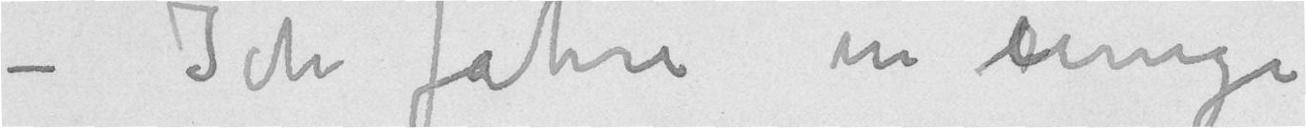– Ich fahre in einige
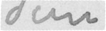denn
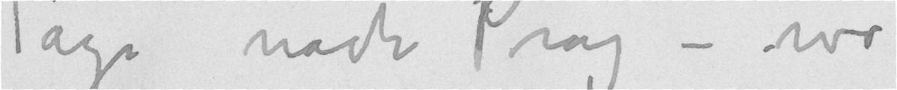Tage nach Prag – wo
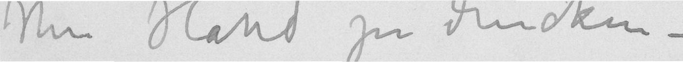Ihre Hand zu drucken –
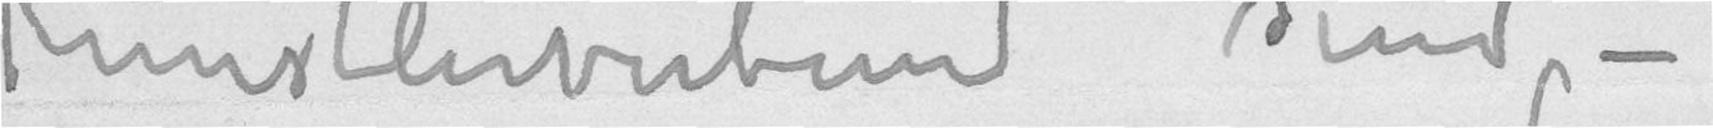Künstlerverbund sind –
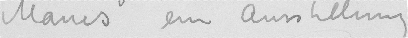Manes» eine Ausstellung
